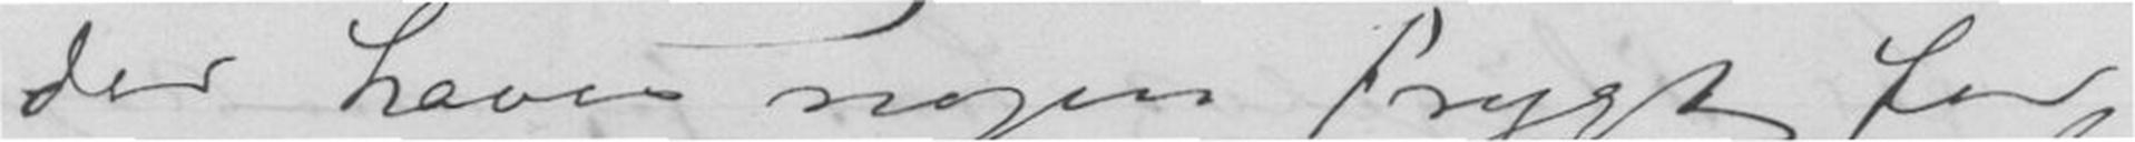der have nogen Frygt for
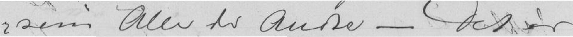som Alle de Andre - Det er
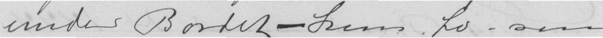under Bordet - kun to som
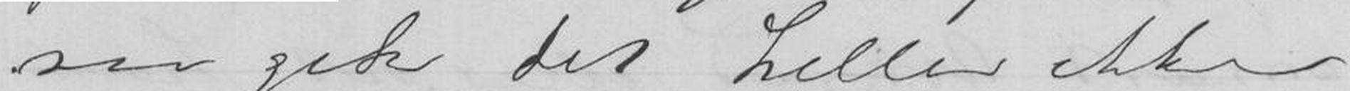saa gik det heller ikke
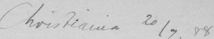Christiania 20/7.88
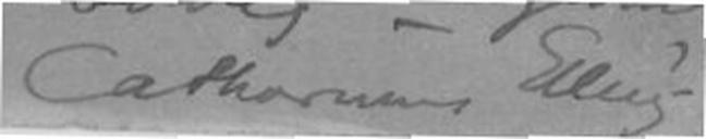Catharinus Elling
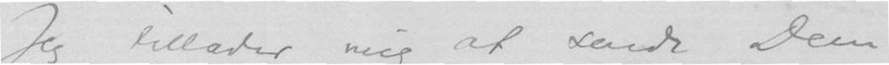Jeg tillader mig at sende Dem
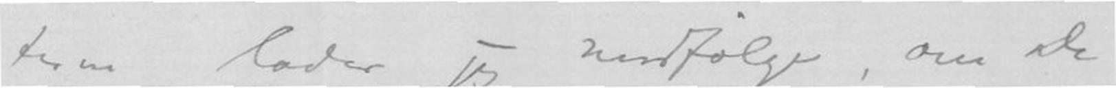terne lader jeg medfølge, om De
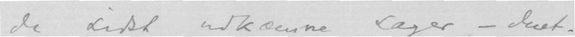de sidste udkomne sager - Duet-
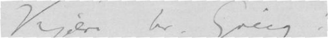Kjære hr. Grieg!
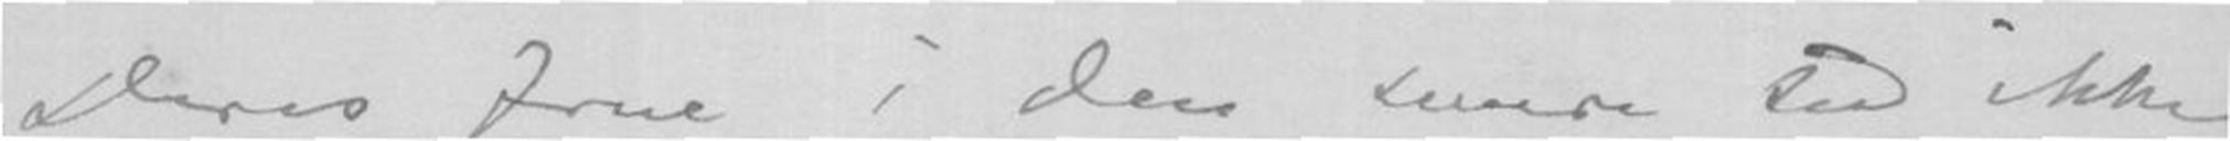Deres frue i den senere tid ikke
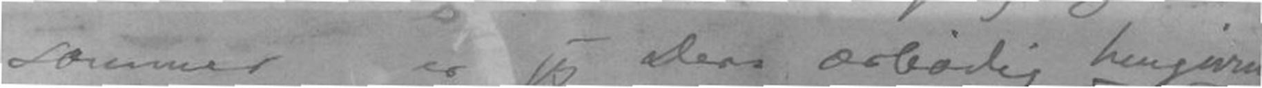sommer er jeg Deres ærbødig hengivne
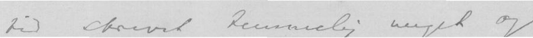tid skrevet temmeligt meget og
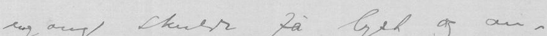engang skulde få lyst og an-
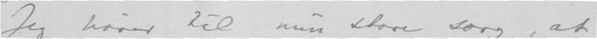Jeg hører til min store sorg, at
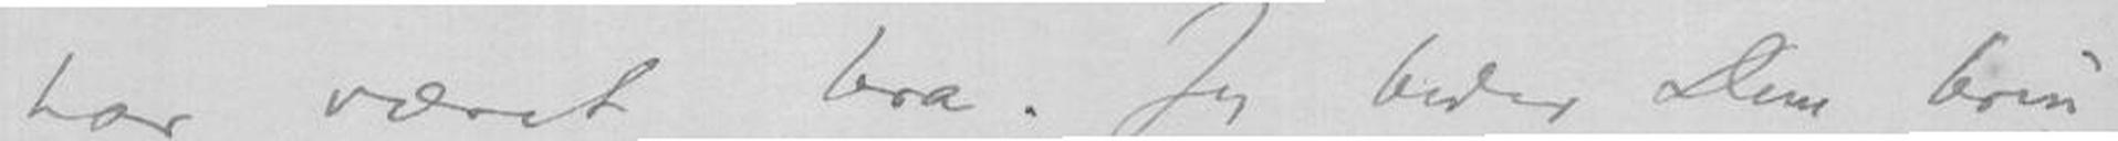har været bra. Jeg beder Dem brin-
